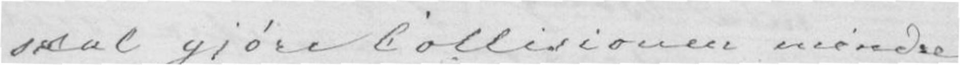skal gjøre Collisionen mindre
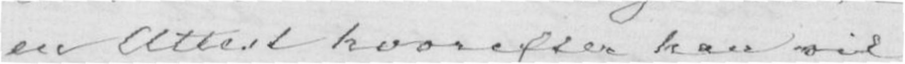en Attest hvorefter han vil
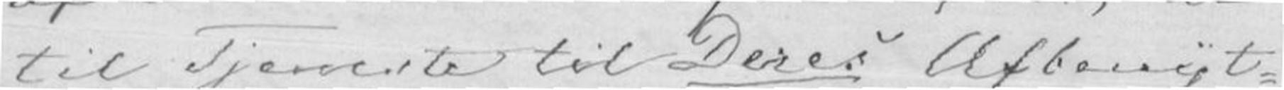til Tjeneste til Deres avbenyt-
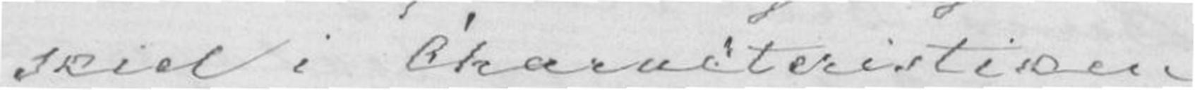skiel i Characteristiken
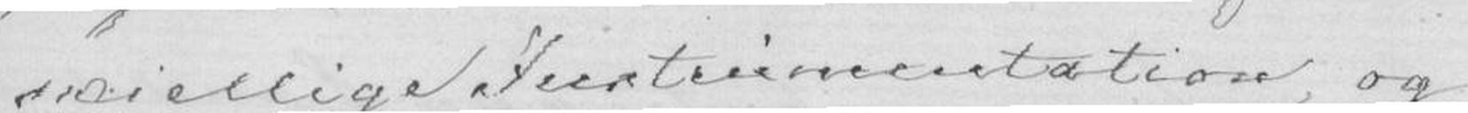skiellige Instrumentation, og
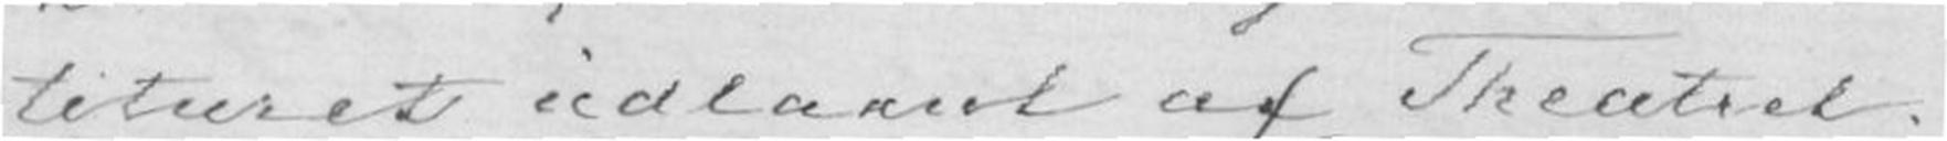tituret udlaant af Theatret.
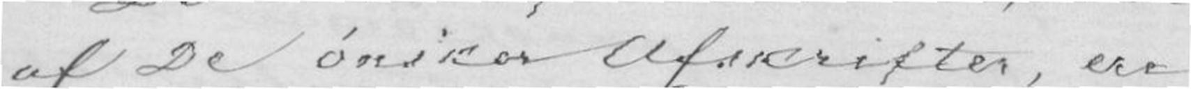af De ønsker Afskrifter, ere
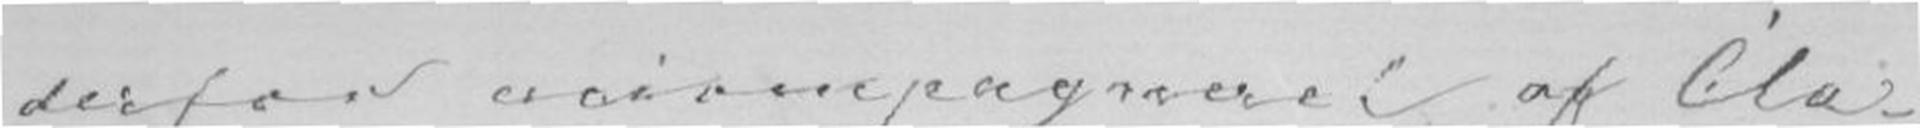derfor compagneret af Cla-
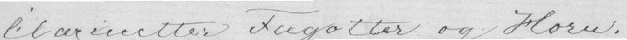Clarinetter Fagotter og Horn.
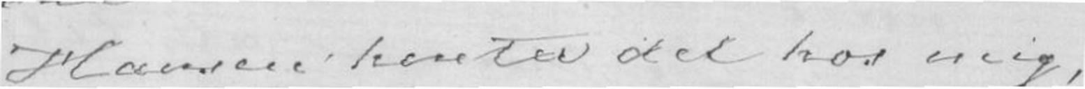Hansen henter det hos mig,
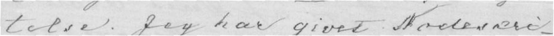telse. Jeg har givet Nodeskri-
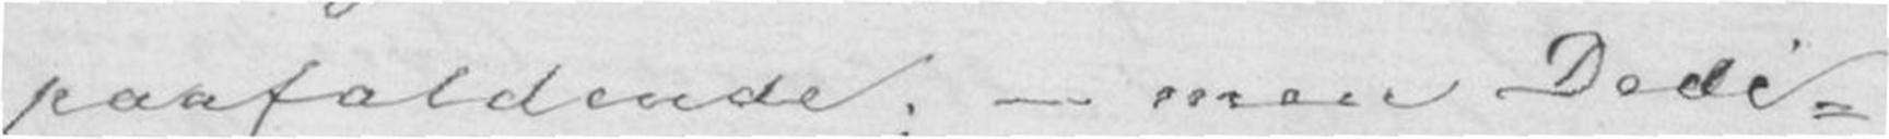paafaldende;- men Dedi-
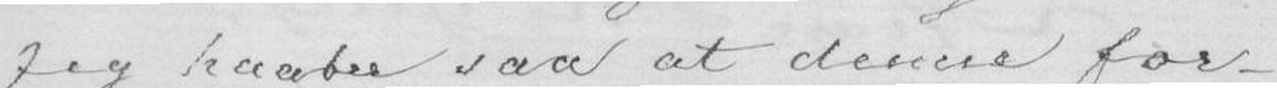Jeg haaber saa at denne for-
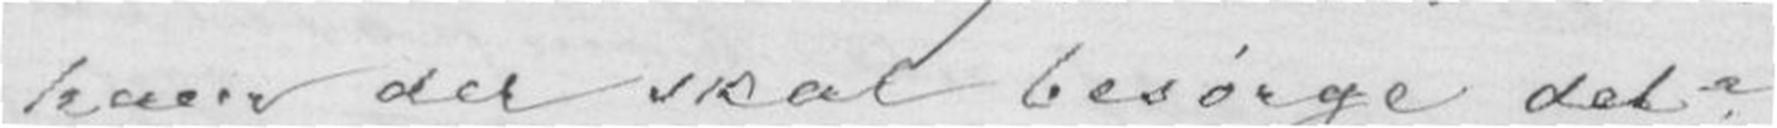han der skal besørge det?-
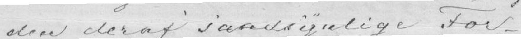den deraf sandsynlige For-
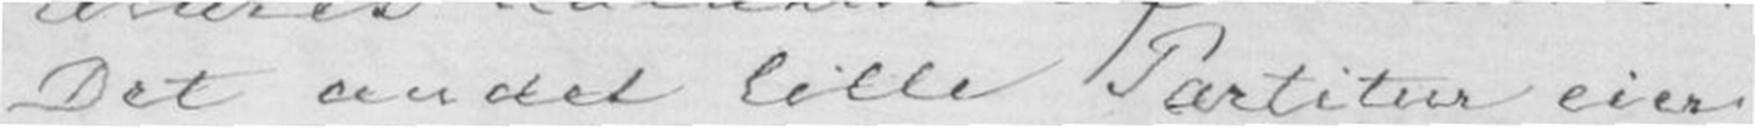Det andet lille Partitur eier
